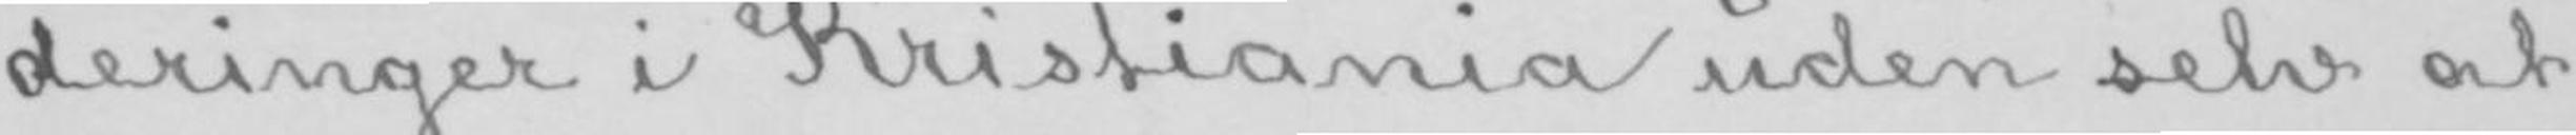deringer i Kristiania uden selv at
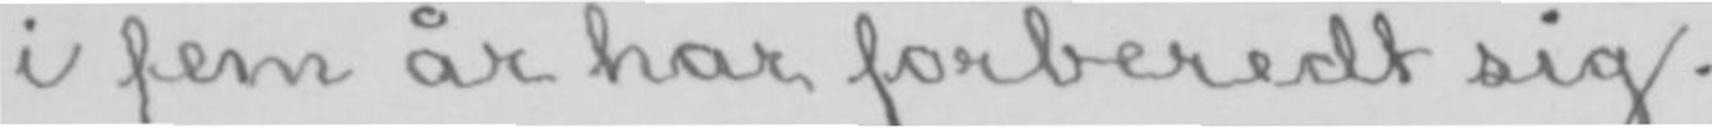i fem år har forberedt sig.
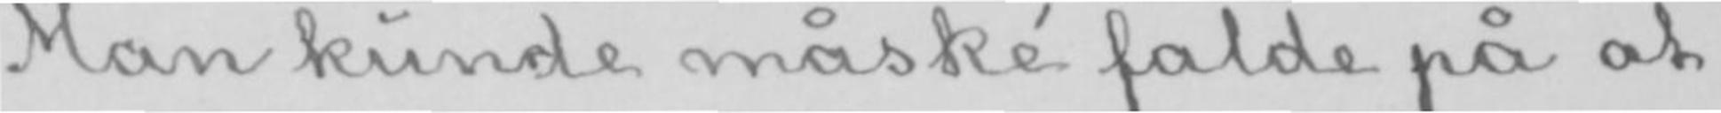Man kunde måské falde på at
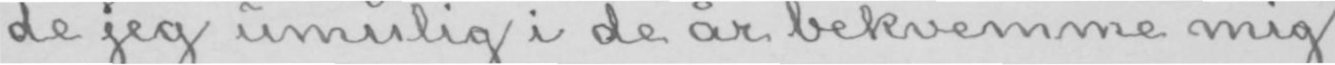de jeg umulig i de år bekvemme mig
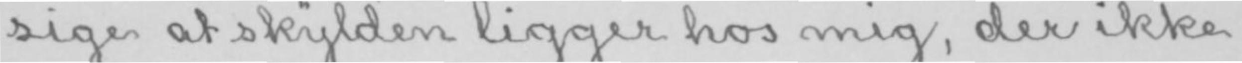sige at skylden ligger hos mig, der ikke
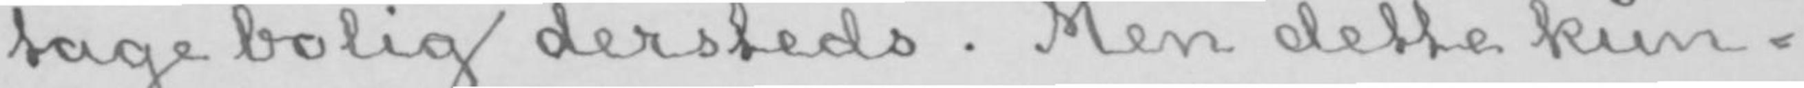tage bolig dersteds. Men dette kun-
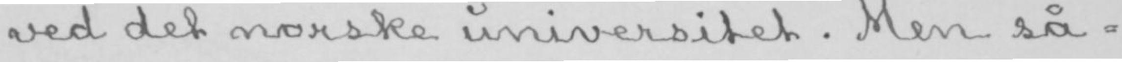ved det norske universitet. Men så-
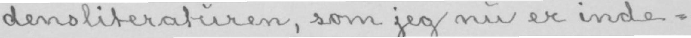densliteraturen, som jeg nu er inde-
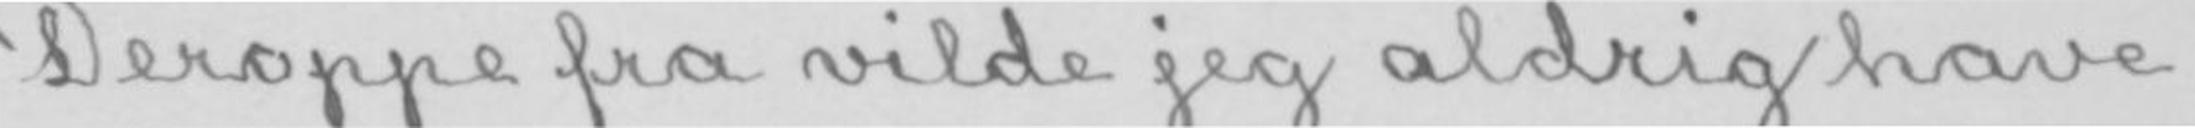Deroppe fra vilde jeg aldrig have
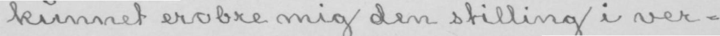kunnet erobre mig den stilling i ver-
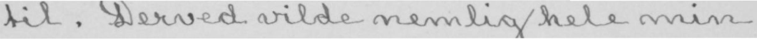til. Derved vilde nemlig hele min
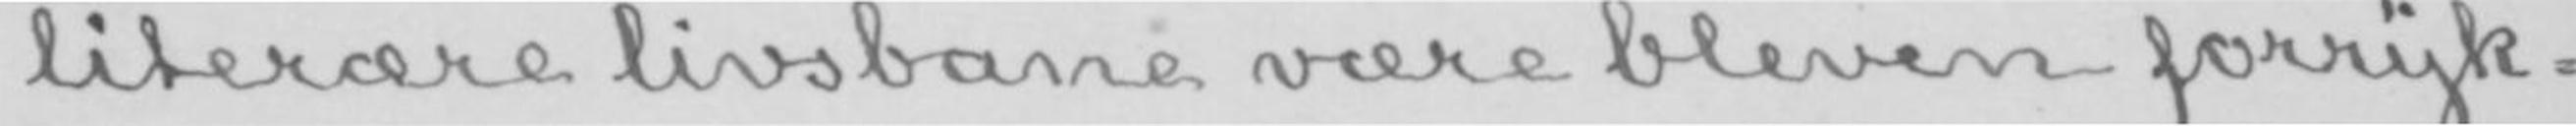literære livsbane være bleven forryk-
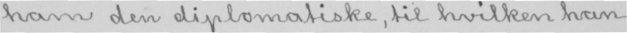ham den diplomatiske, til hvilken han
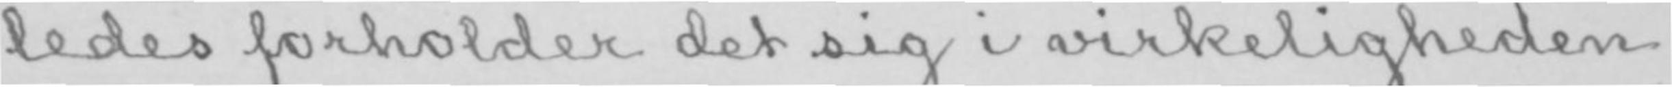ledes forholder det sig i virkeligheden
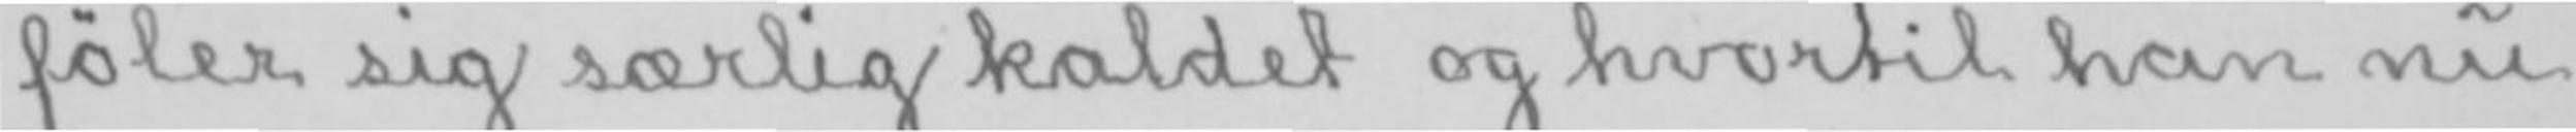føler sig særlig kaldet og hvortil han nu
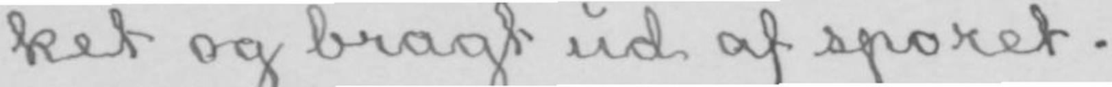ket og bragt ud af sporet.
